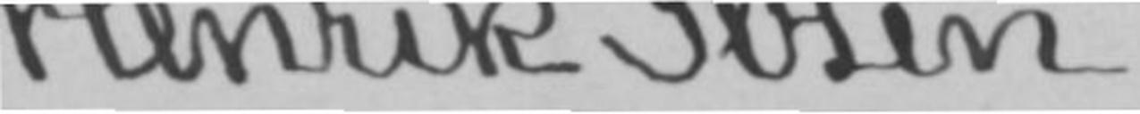Henrik Ibsen.
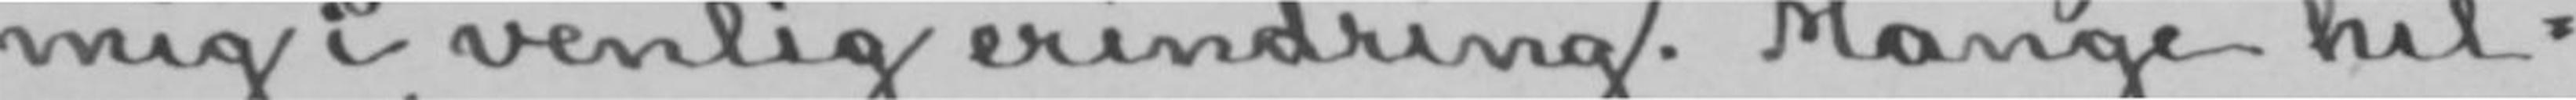mig i venlig erindring. Mange hil-
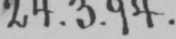24.3.94.
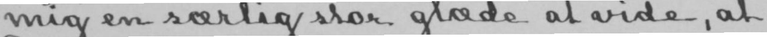mig en særlig stor glæde at vide, at
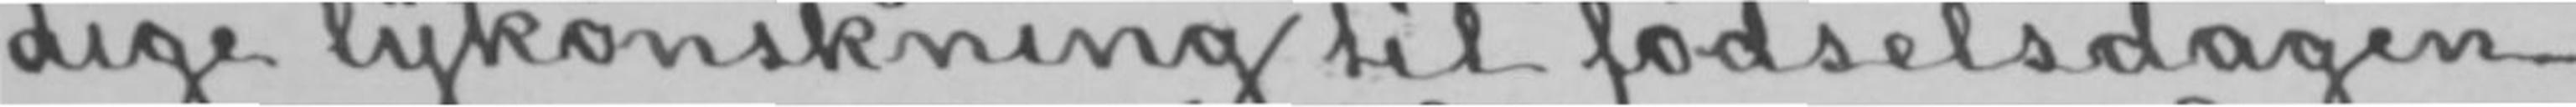dige lykønskning til fødselsdagen
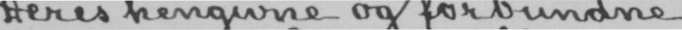Deres hengivne og forbundne
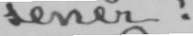sener!
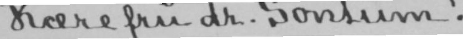Kære fru dr. Sontum!
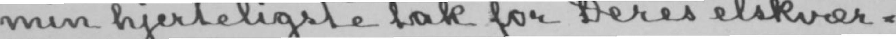min hjerteligste tak for Deres elskvær-
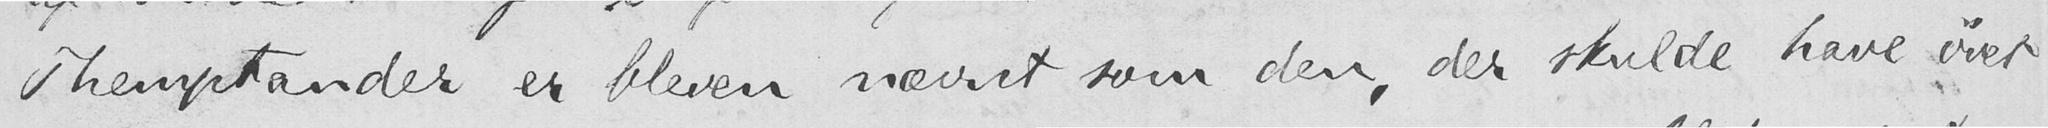Themptander er bleven nævnt som den, der skulde have øvet
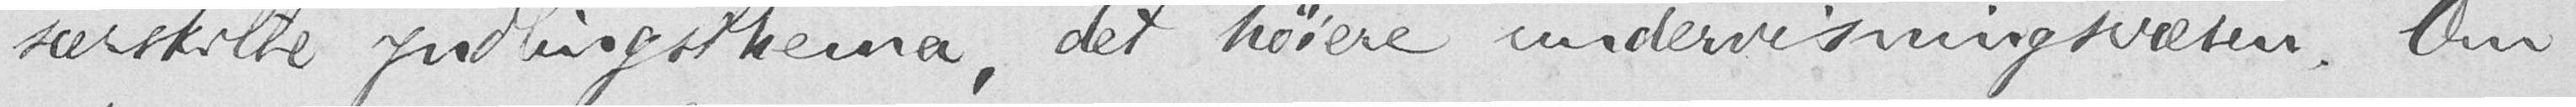særskilte yndlingsthema, det høiere undervisningsvæsen. Om
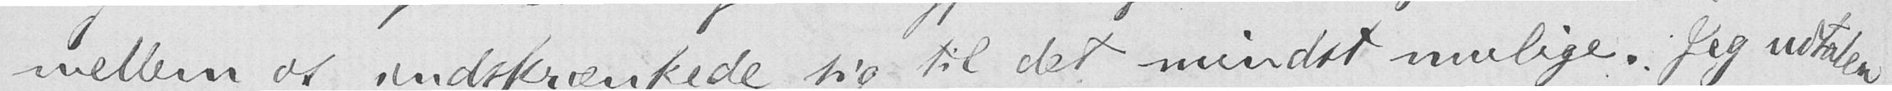mellem os indskrænkede sig til det mindst mulige. Jeg udtaler
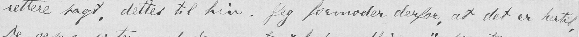rettere sagt, dettes til hin. Jeg formoder derfor, at det er hertil,
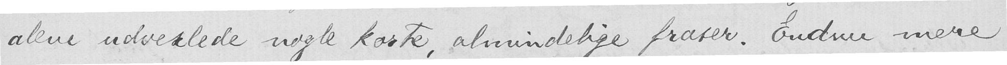alene udvexlede nogle korte, almindelige fraser. Endnu mere
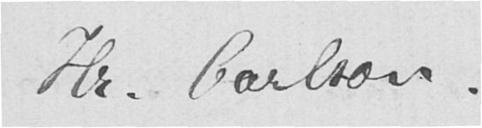Hr. Carlson.
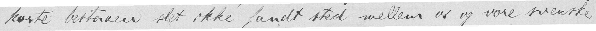korte bestaaen slet ikke fandt sted mellem os og vore svenske
